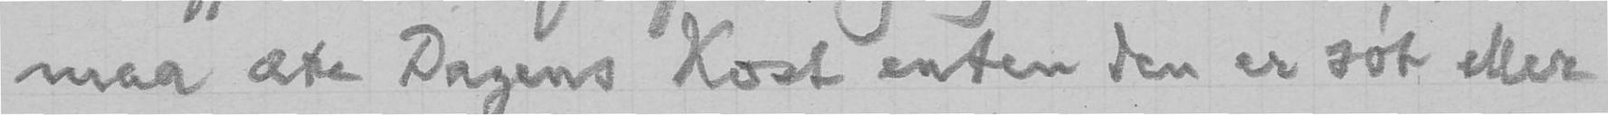maa æte Dagens Kost enten den er søt eller
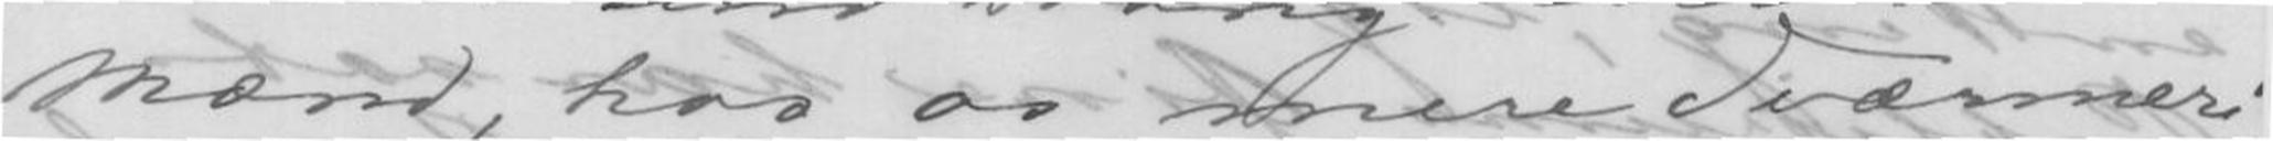Mænd, hos os sværmeri.
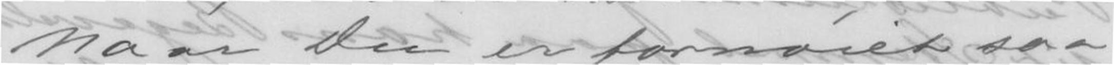Naar Du er fornøiet, saa
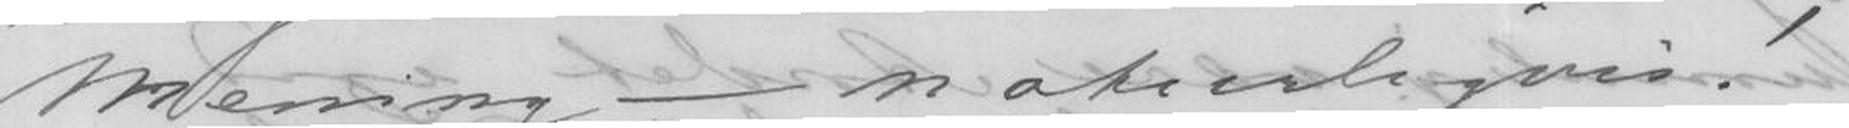Mening - naturligvis!
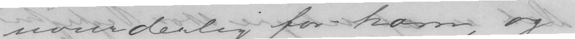uvurderlig for - ham, og
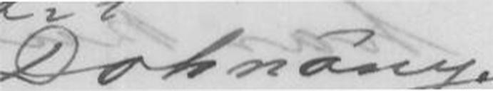Dohnâny.
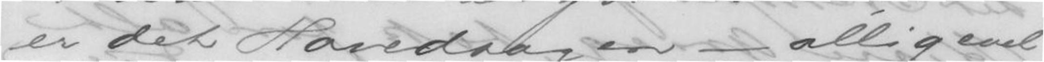er det Hovedsagen - alligevel
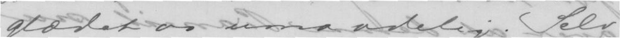glædet os umaadelig. Selv
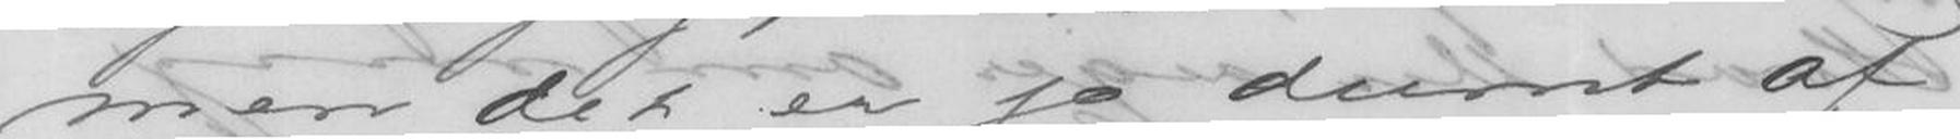men det er jo dumt af
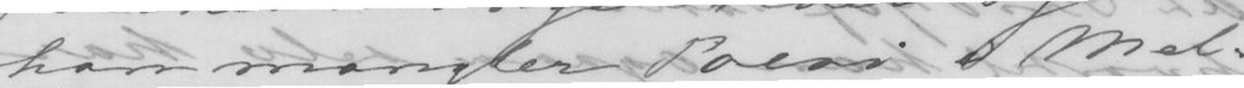han mangler Poesi i Mel-
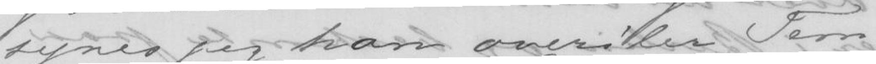synes jeg han overiler Tem-
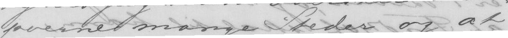poerne mange Steder og at
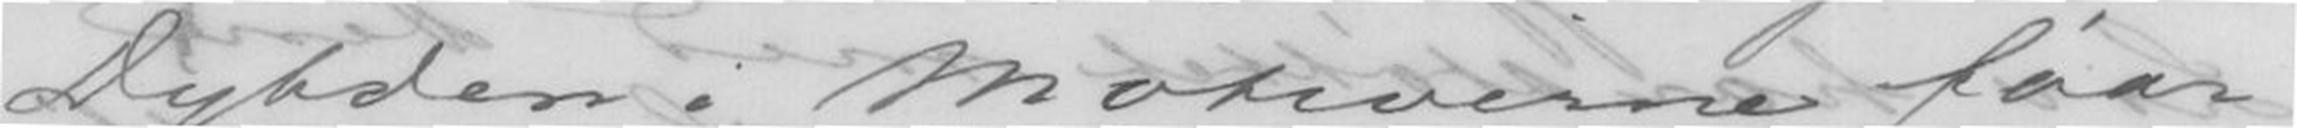Dybden. Motiverne faar
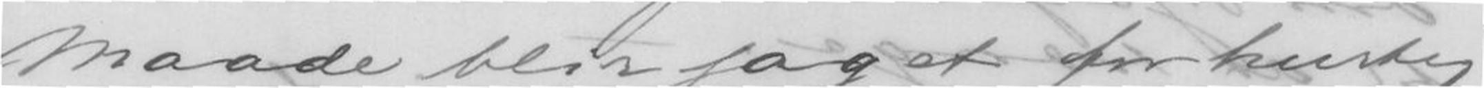Maade blir jaget for hurtig
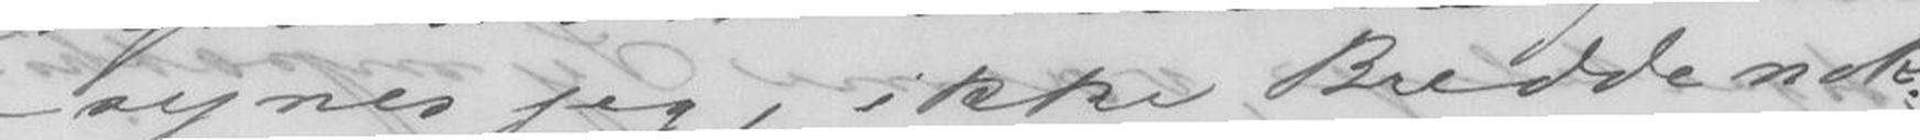- synes jeg, ikke Bredde nok;
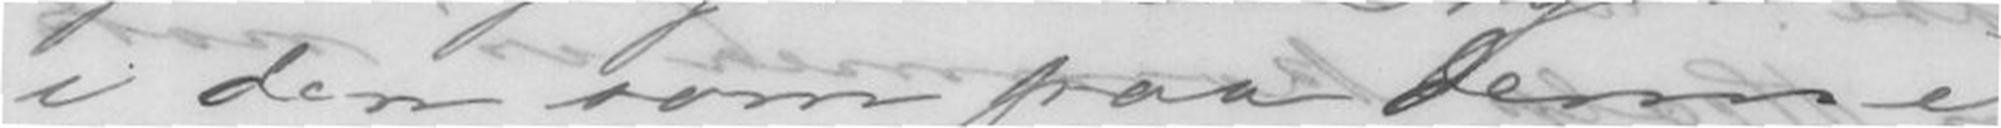i den som paa denne
